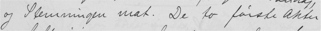og Stemningen mat. De to første Akter
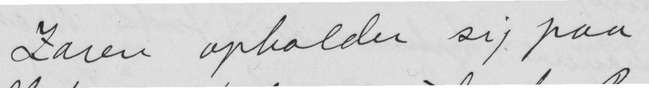Zaren opholder sig paa
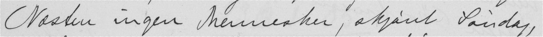Næsten ingen Mennesker, skjønt Søndag,
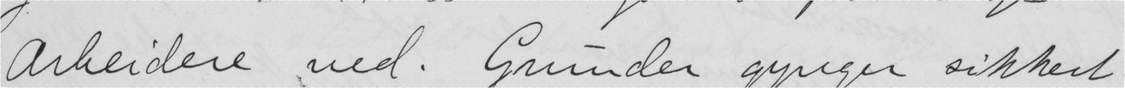Arbeidere ned. Grunden gynger sikkert
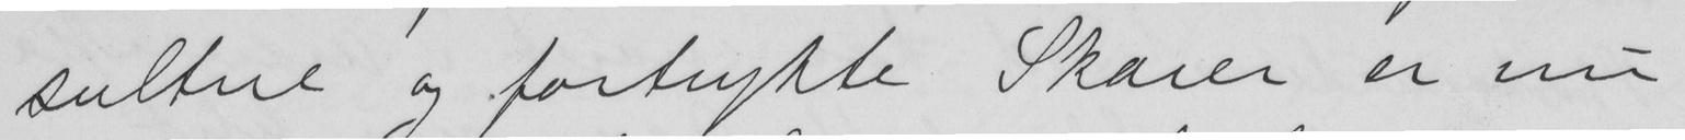sultne og fortrykte Skarer er nu
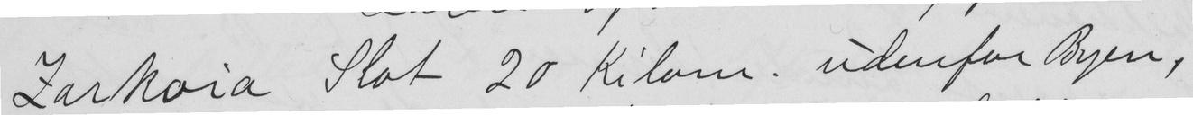Zarkoia Slot 20 Kilom. udenfor Byen,
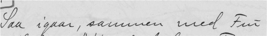Saa igaar, sammen med Fru
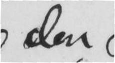den
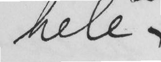hele
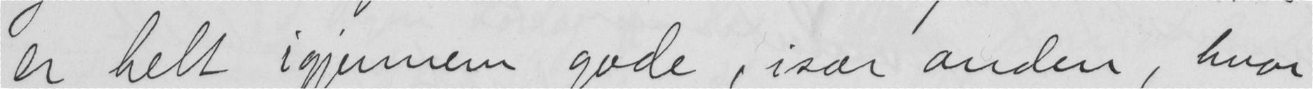er helt igjennem gode, især anden, hvor
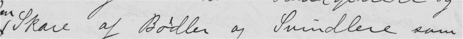skare af Bødler og Svindlere som
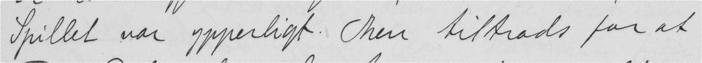Spillet var ypperligt. Men tiltrods for at
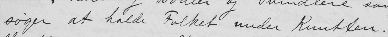søger at holde Folket under Knutten.
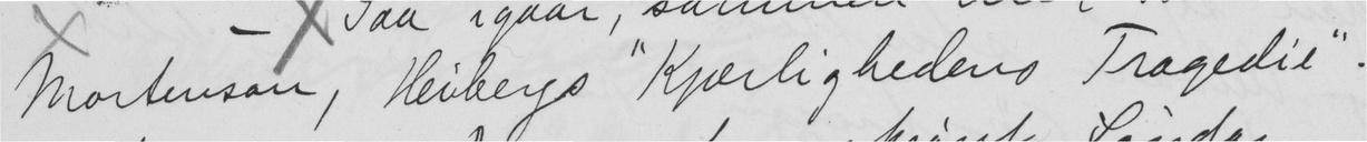Mortenson, Heibergs "Kjærlighedens Tragedie"
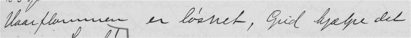Vaarflommen er løsnet. Gud hjælpe det
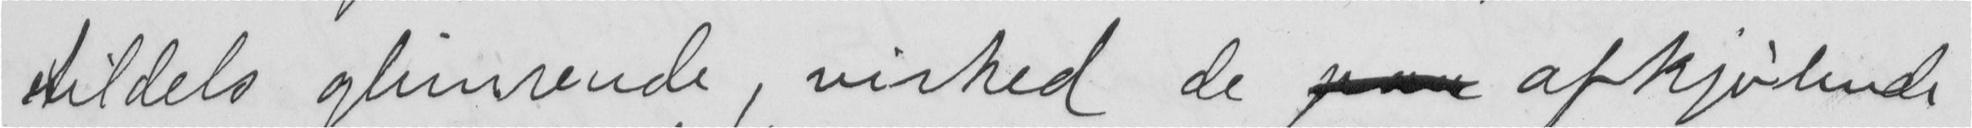tildels glimrende, virked de  afkjølende
